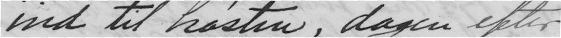ind til høsten, dagen efter
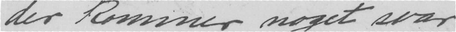der kommer noget svar
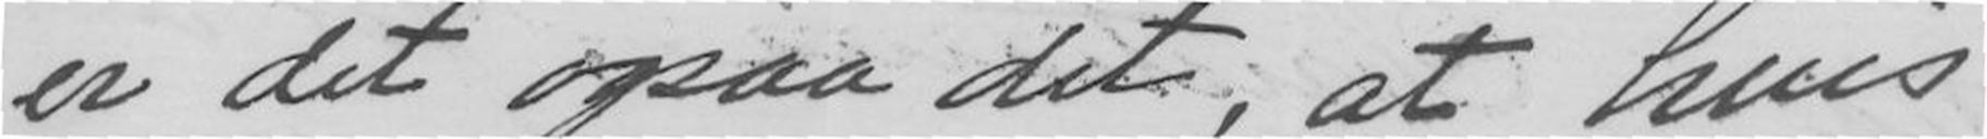er det ogsaa det, at hvis
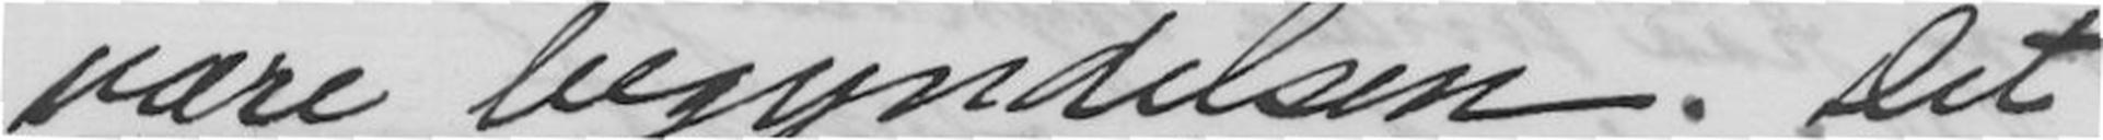være begyndelsen. Det
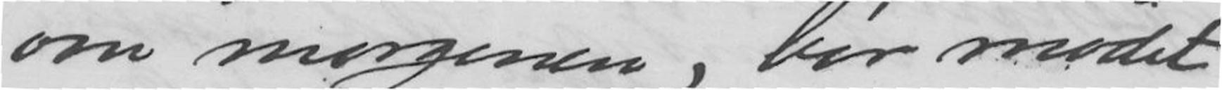om morgenen, bør mødet
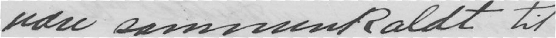være sammenkaldt til
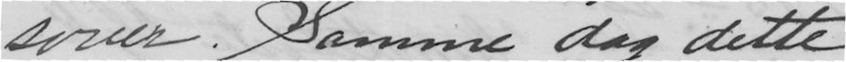sover. Samme dag dette
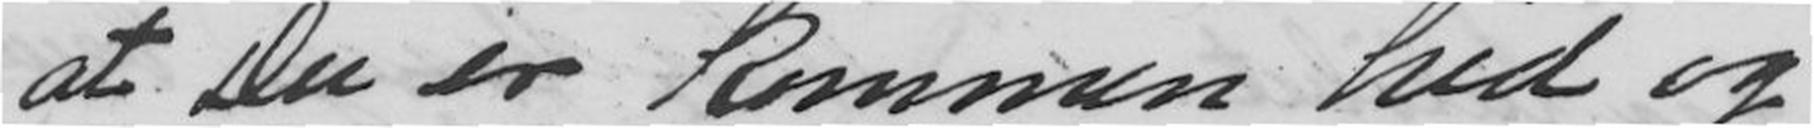at Du er kommen hid og
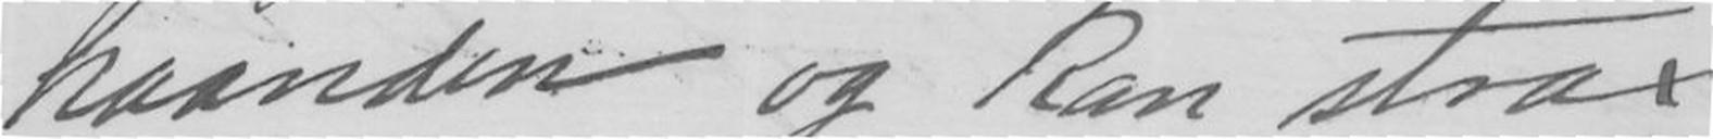haanden og kan strax
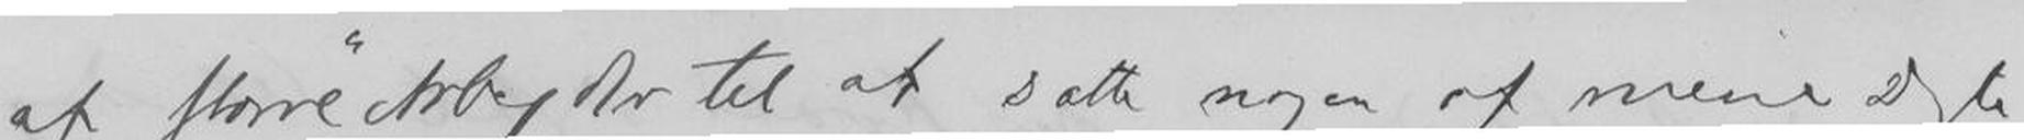af større Arbejder til at sætte nogen af mine Digte
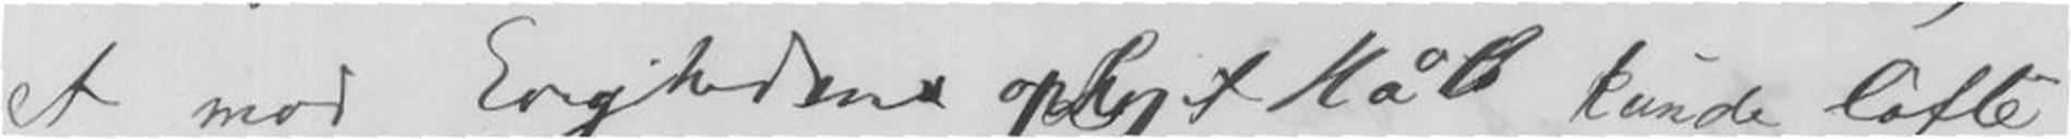et mod Evighedene ophøjet Håb kunde Løfte
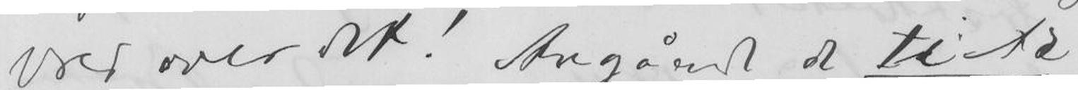vred over det! Angående de ti År
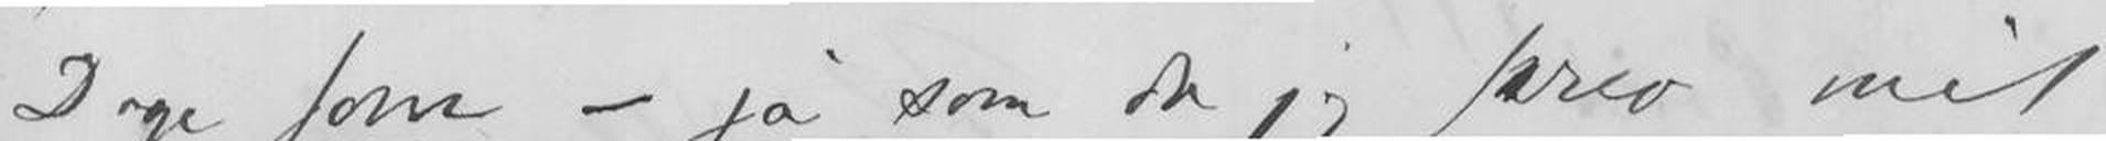Dage som - ja som da jeg skrev mit
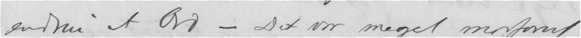endnu et Ord - Det var meget morsomt
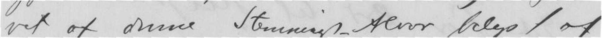vet af denne Stemnings Alvor belyst af
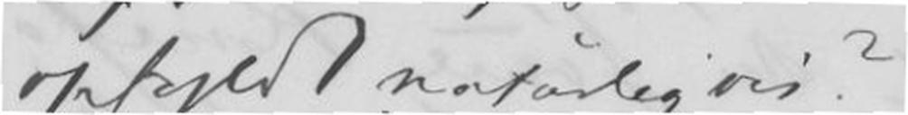opfyldt naturligvis?
